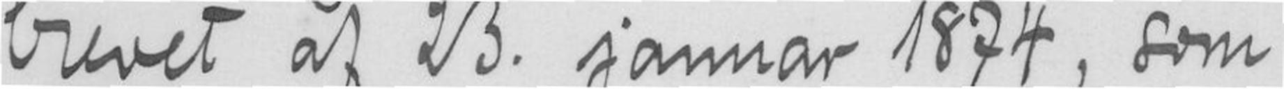brevet af 23 januar 1874, som
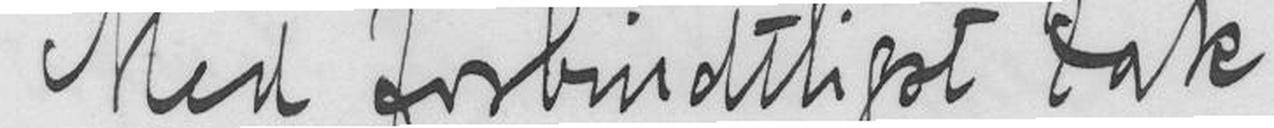Med forbindtligst tak
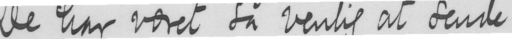De har været så venlig at sende
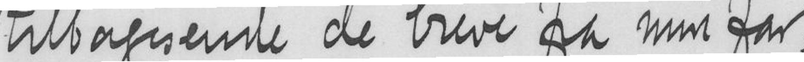tilbagesende de Breve fra min far,
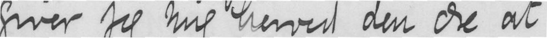giver jeg herved den ære at
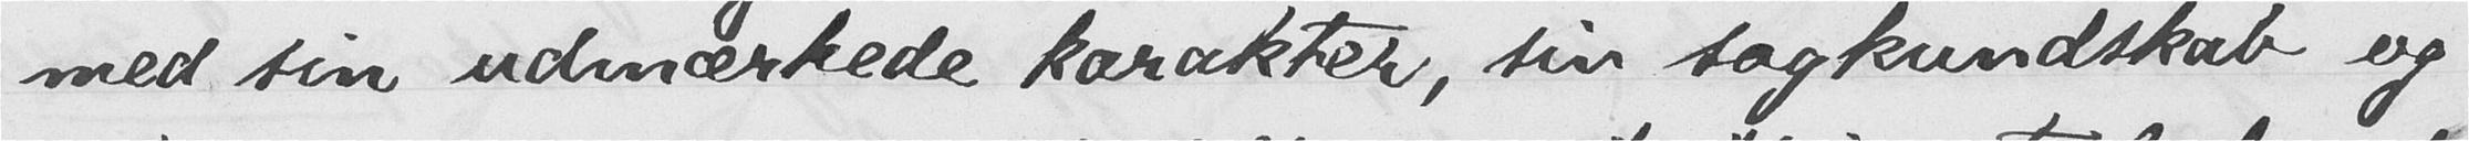med sin udmærkede karakter, sin sagkundskab og
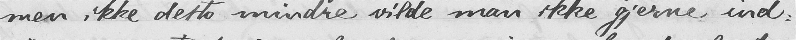men ikke desto mindre vilde man ikke gjerne ind-
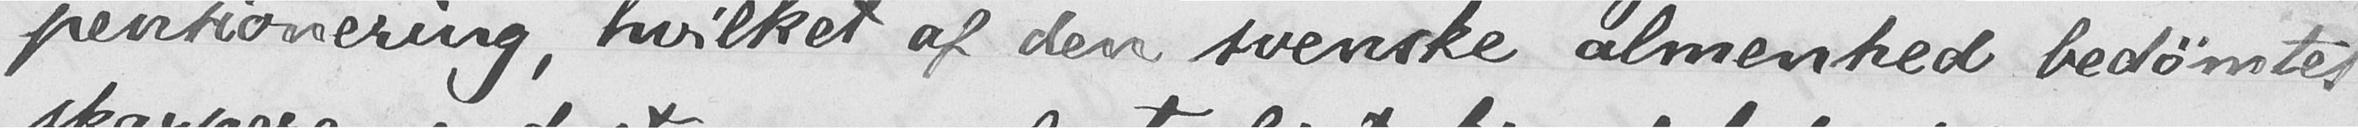pensionering, hvilket af den svenske almenhed bedømtes
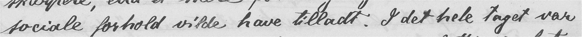sociale forhold vilde have tilladt. I det hele taget var
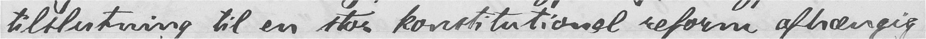tilslutning til en stor konstitutionel reform afhængig
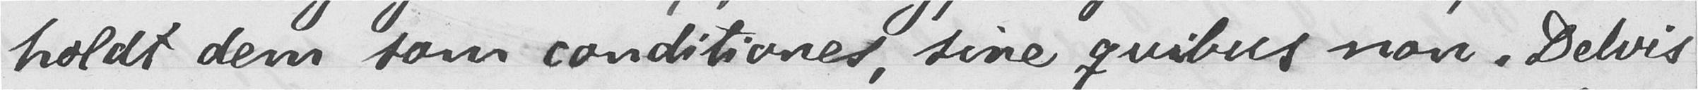holdt dem som conditiones, sine quibus non. Delvis
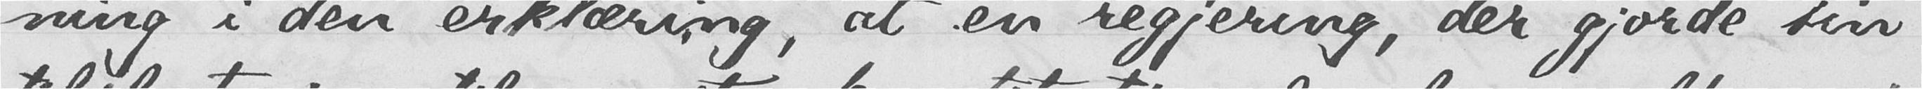ning i den erklæring, at en regjering, der gjorde sin
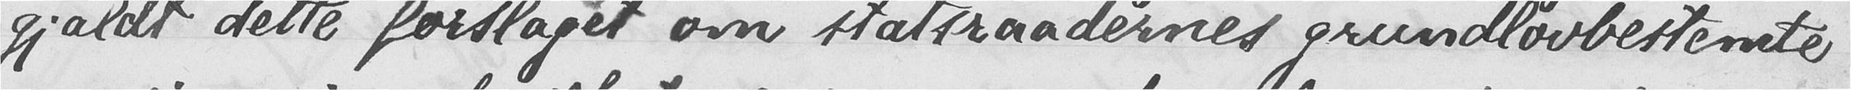gjaldt dette forslaget om statsraadernes grundlovbestemte
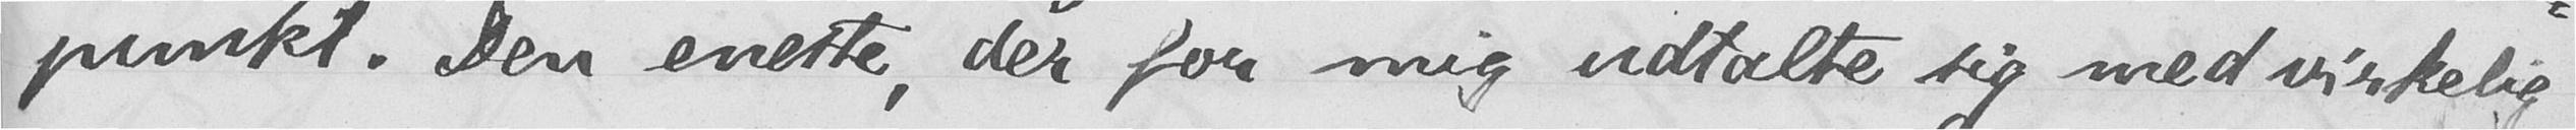punkt. Den eneste, der for mig udtalte sig med virkelig
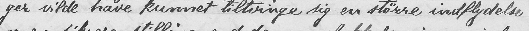ger vilde have kunnet tiltvinge sig en større indflydelse
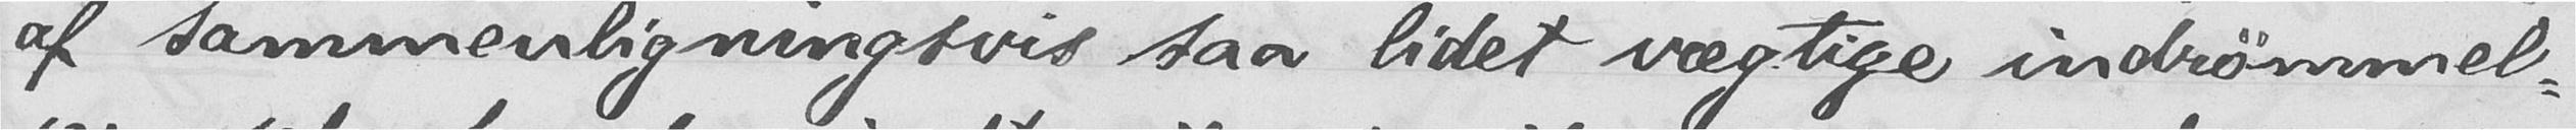af sammenligningsvis saa lidet vægtige indrømmel-
